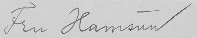Fru Hamsun
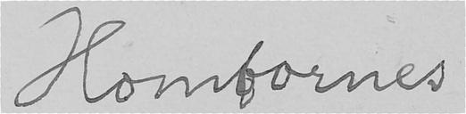Hombornes
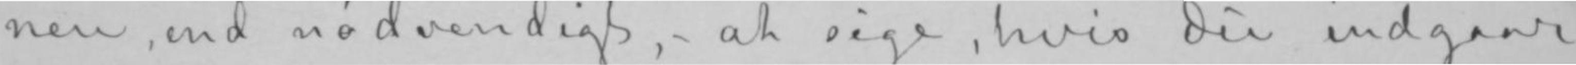nen, end nødvendigt,- at sige, hvis Du indgaar
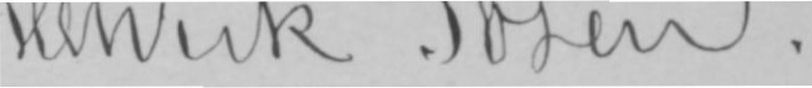Henrik Ibsen.
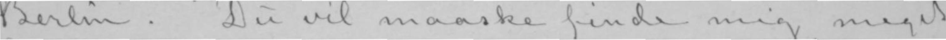Berlin. Du vil maaske finde mig meget
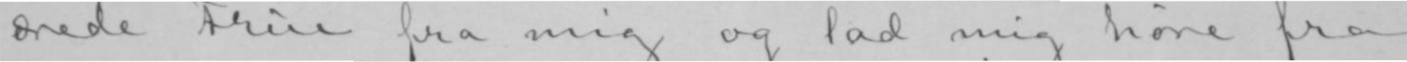ærede Frue fra mig og lad mig høre fra
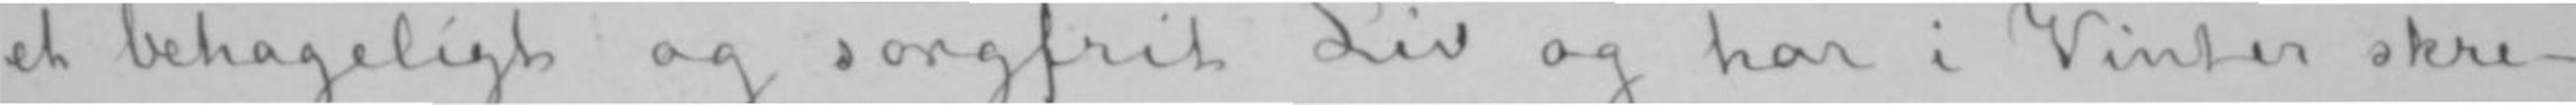et behageligt og sorgfrit Liv og har i Vinter skre-
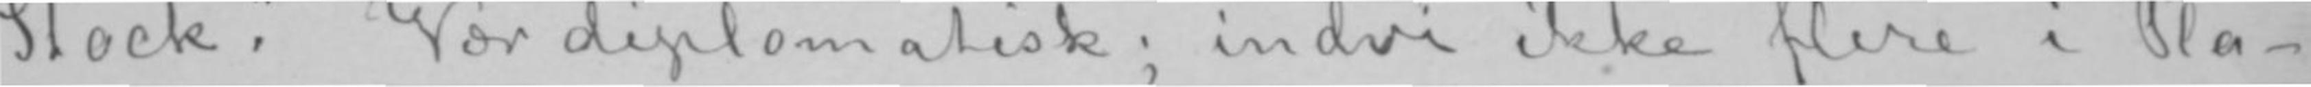Stock». Vær diplomatisk; indvi ikke flere i Pla-
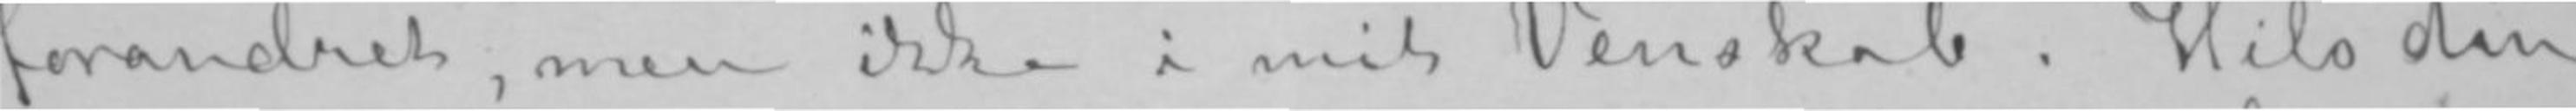forandret, men ikke i mit Venskab. Hils din
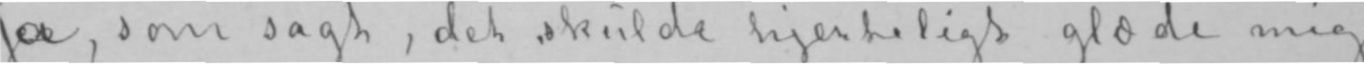JaJe, som sagt, det skulde hjerteligt glæde mig
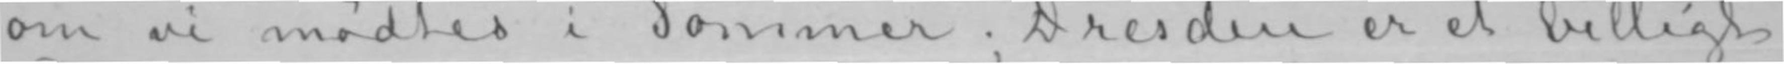om vi mødtes i Sommer; Dresden er et billigt
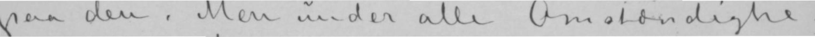paa den. Men under alle Omstændighe-
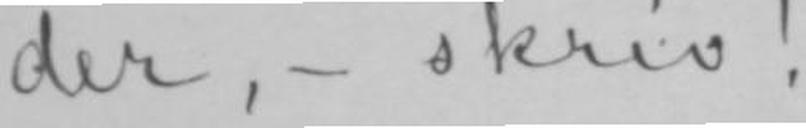der,- skriv!
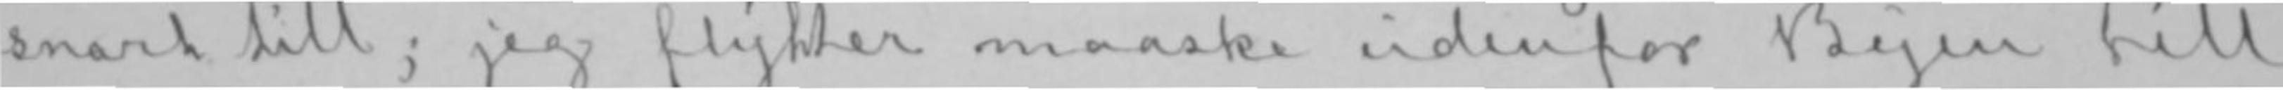snart till; jeg flytter maaske udenfor Byen till
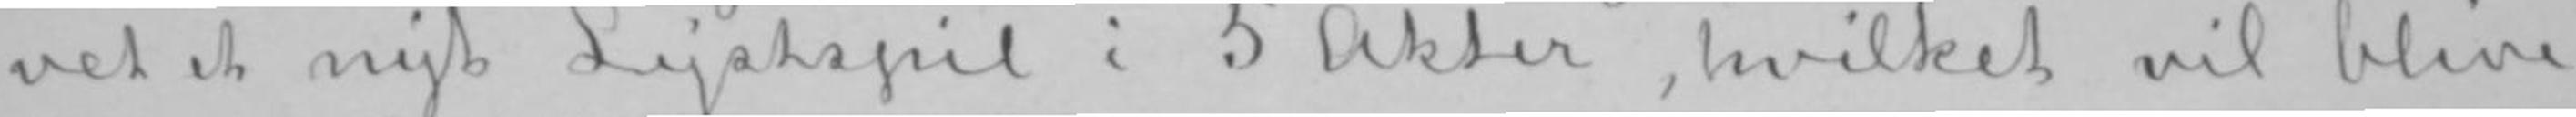vet et nyt Lystspil i 5 Akter, hvilket vil blive
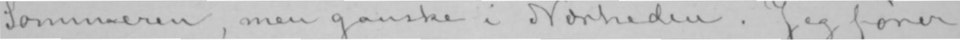Sommeren, men ganske i Nærheden. Jeg fører
